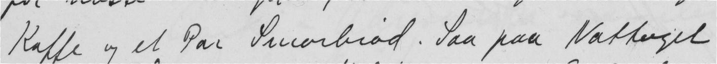Kaffe og et Par Smørbrod. Saa paa Nattoget
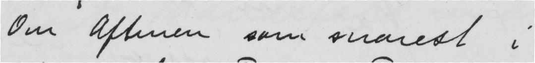Om Aftenen som snarest i
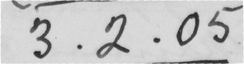3.2.05
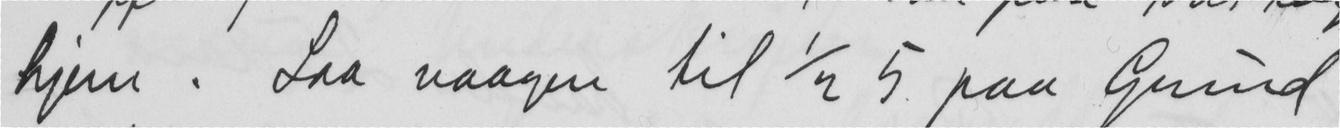hjem. Laa vaagen til ½ 5 paa Grund
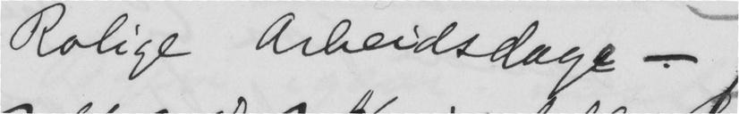Rolige Arbeidsdage -
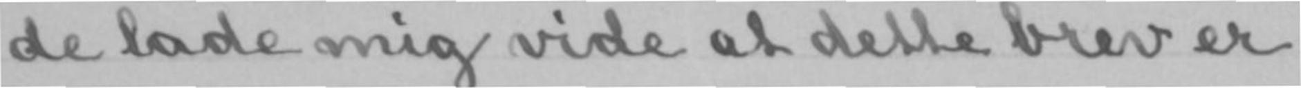de lade mig vide at dette brev er
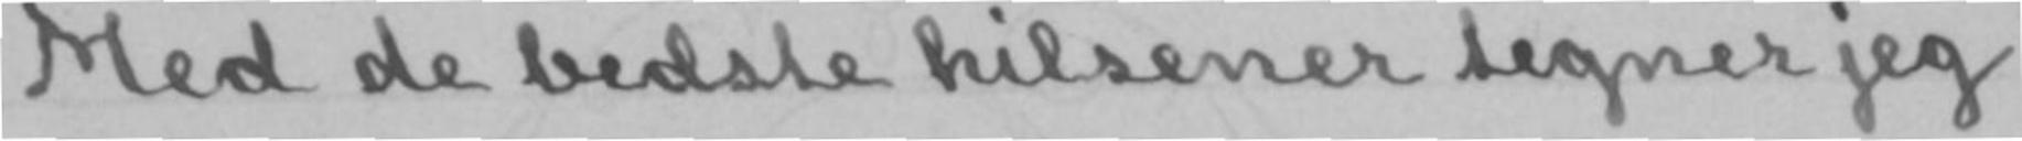Med de bedste hilsener tegner jeg
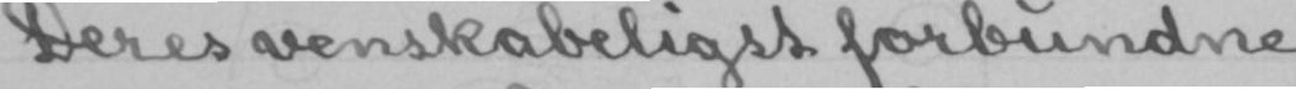Deres venskabeligst forbundne
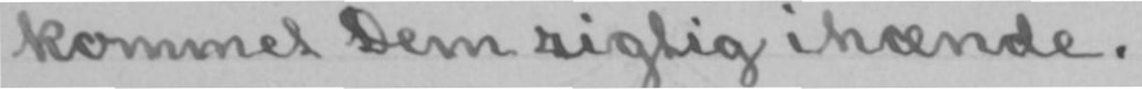kommet Dem rigtig ihænde.
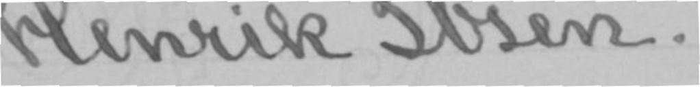Henrik Ibsen.
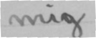mig
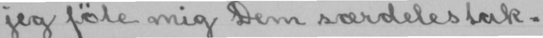jeg føle mig Dem særdeles tak-
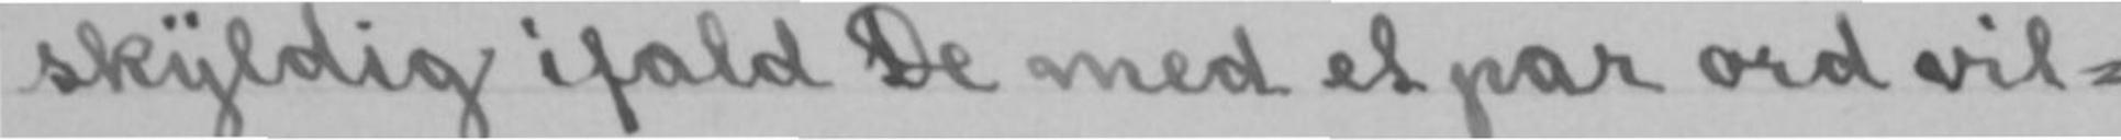skyldig ifald De med et par ord vil-
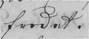fredet.
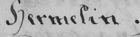Hermelin.
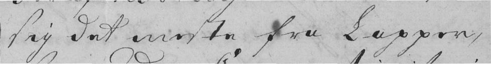sig det meste fra Lapper,
